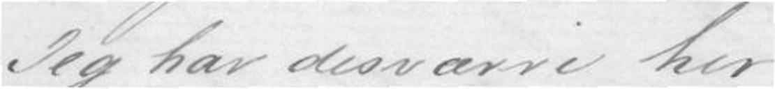Jeg har desværre her
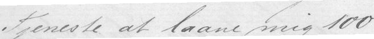Tjeneste at laane mig 100
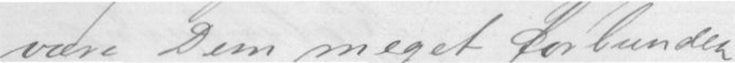være Dem meget forbunden
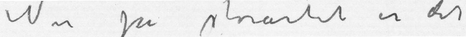Nu ja storarted er det
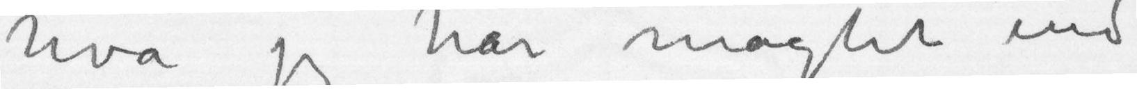hva jeg har magtet end
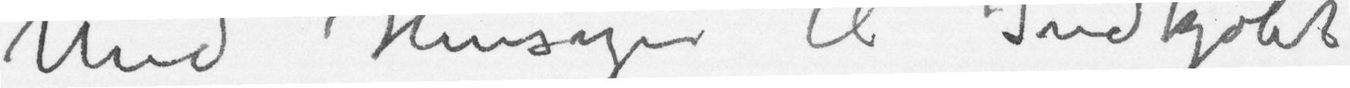Med Hensyn til Indkjøbet
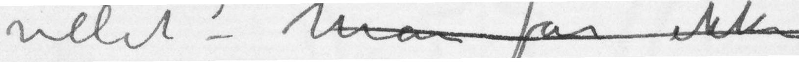villet – Man får ikke
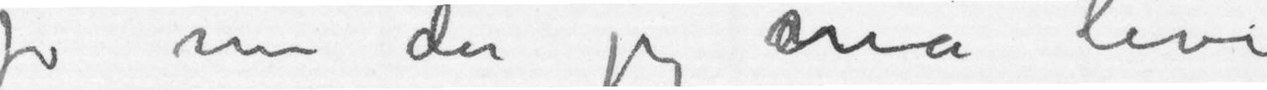jo nu da jeg må leve
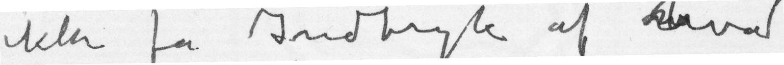ikke få Indtryk af hvad
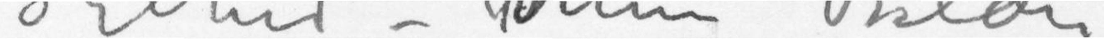Stilhed – Mine Bilder
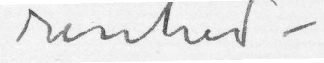renhed –
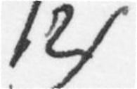12)
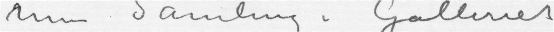min Samling i Galleriet
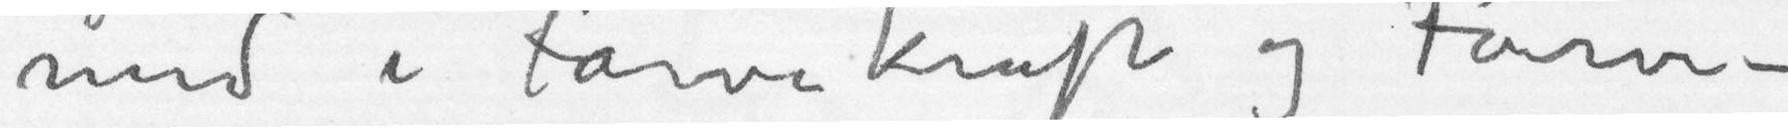med i Farvekraft og Farve-
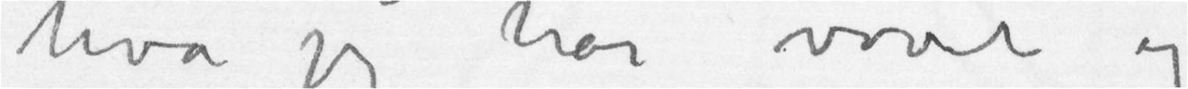hva jeg har vovet og
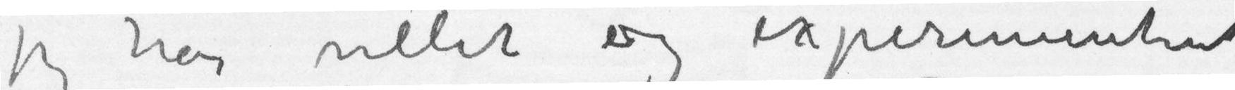jeg har villet og experimenteret
